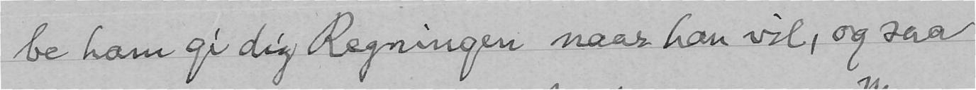be ham gi dig Regningen naar han vil, og saa
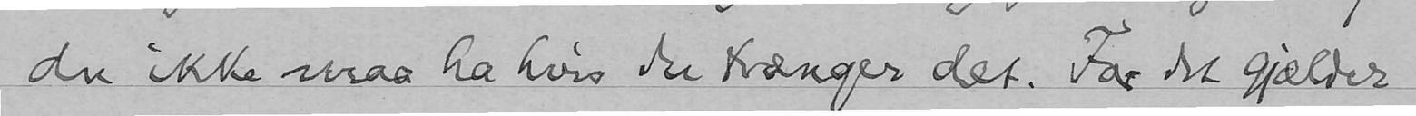du ikke maa ha hvis du trænger det. For det gjælder
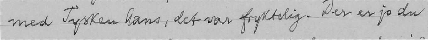med Tysken hans, det var fryktelig. Der er jo du
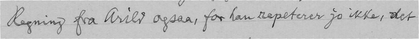Regning fra Arild ogsaa, for han repeterer jo ikke, det
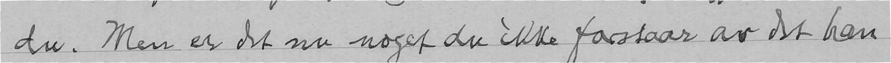du. Men er det nu noget du ikke forstaar av det han
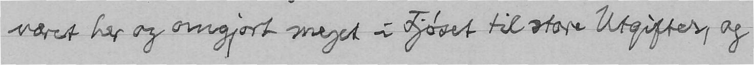været her og omgjort meget i Fjøset til store Utgifter, og
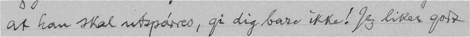at han skal utspørres, gi dig bare ikke! Jeg liker godt
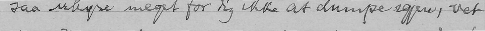saa uhyre meget for dig ikke at dumpe igjen, vet
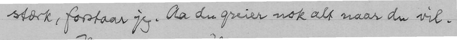stærk, forstaar jeg. Aa du greier nok alt naar du vil.
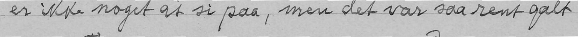er ikke noget at si paa, men det var saa rent galt
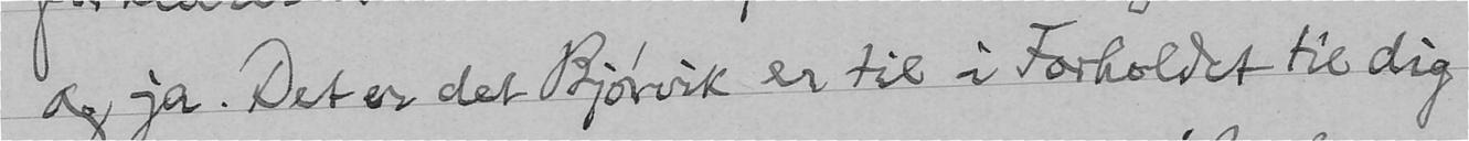og ja. Det er det Bjørvik er til i Forholdet til dig
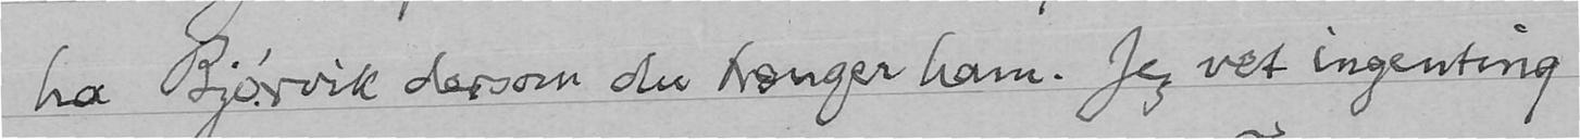ha Bjørvik dersom du trænger ham. Jeg vet ingenting
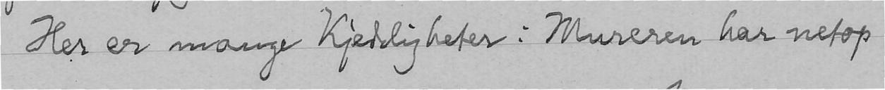Her er mange Kjedeligheter: Mureren har netop
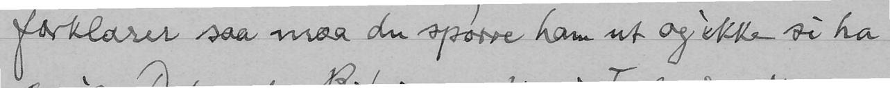forklarer saa maa du spørre ham ut og ikke si ha
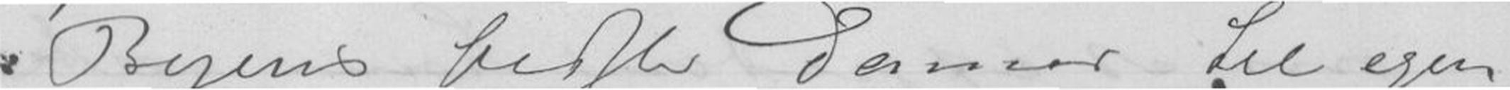Byens bedste Damer til egen
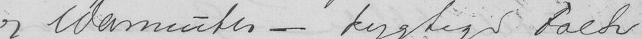og Warmuth - Dygtige Folk
